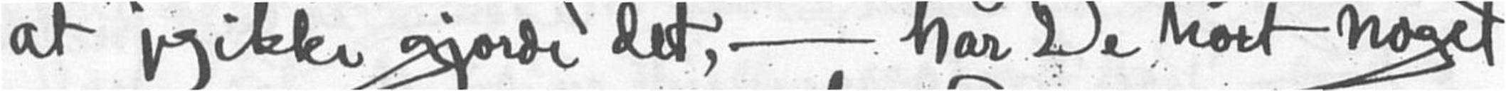at jeg ikke gjorde det, - har De hørt noget
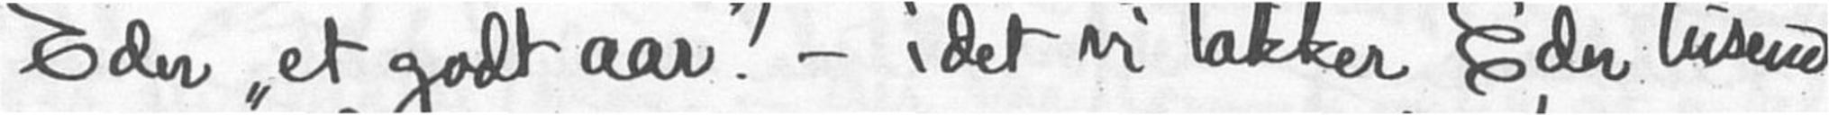Eder "et godt aar"!- idet vi takker Eder tusend
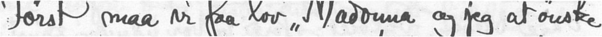Først maa vi faa lov "Madonna" og jeg at ønske
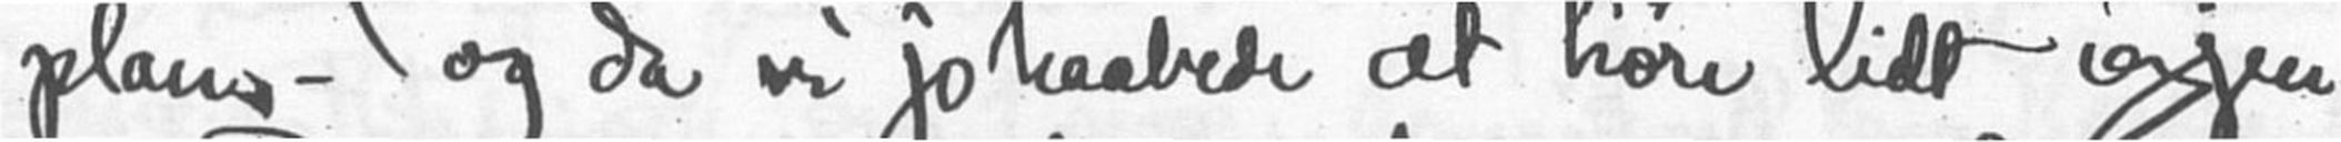plan - og da vi jo haabede at høre lidt igjen
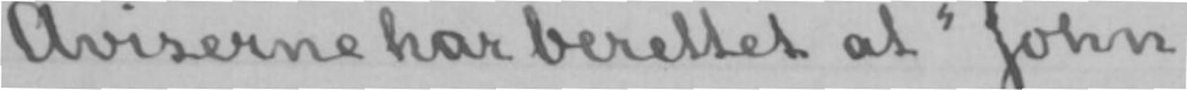Aviserne har berettet at «John
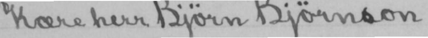Kære herr Bjørn Bjørnson,
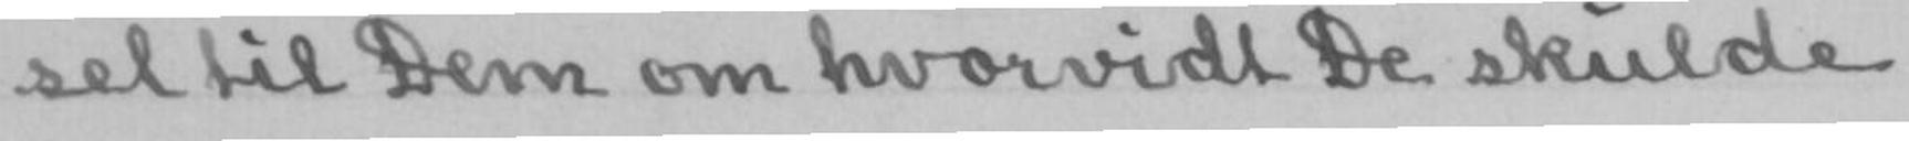sel til Dem om hvorvidt De skulde
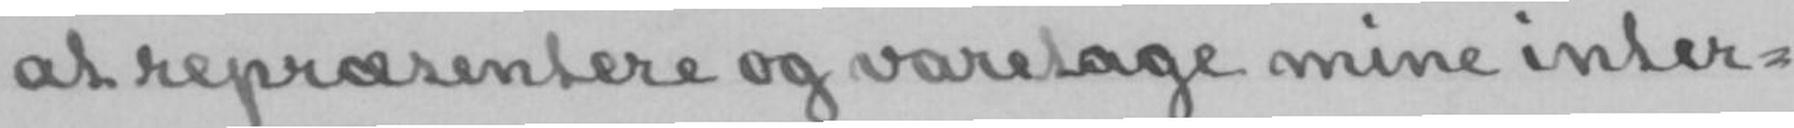at repræsentere og varetage mine inter-
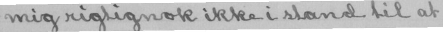mig rigtignok ikke i stand til at
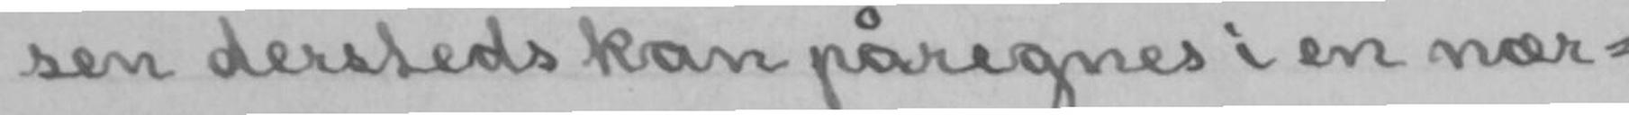sen dersteds kan påregnes i en nær-
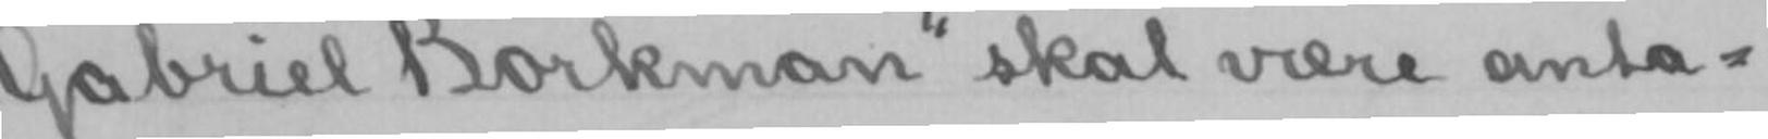Gabriel Borkman» skal være anta-
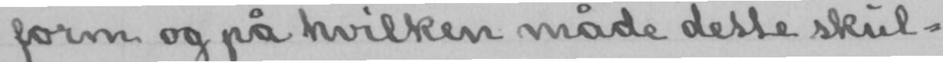form og på hvilken måde dette skul-
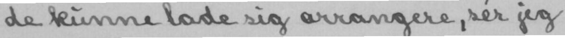de kunne lade sig arrangere, sér jeg
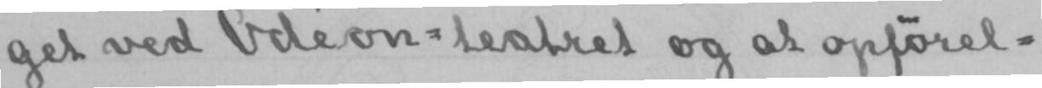get ved Odéon-teatret og at opførel-
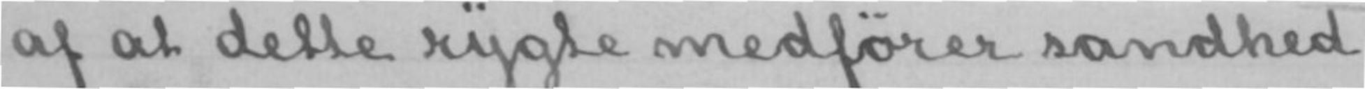af at dette rygte medfører sandhed
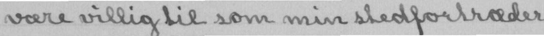være villig til som min stedfortræder
